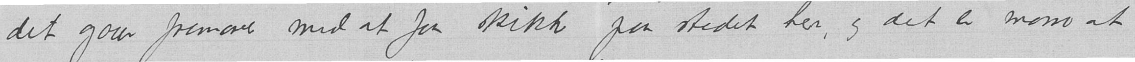det gaar fremover med at faa skikk paa stedet her, og det er morro at
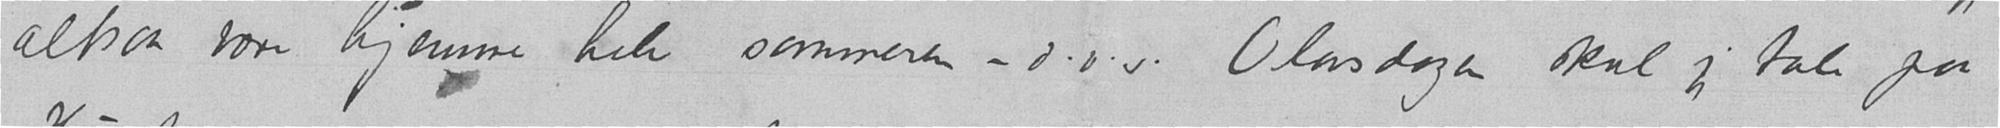altsaa være hjemme hele sommeren – d.v.s. Olavsdagen skal jeg tale paa
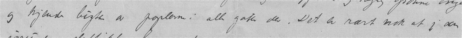og kjende lugten av poplerne i alle gater der. Det er rart nok at jeg som
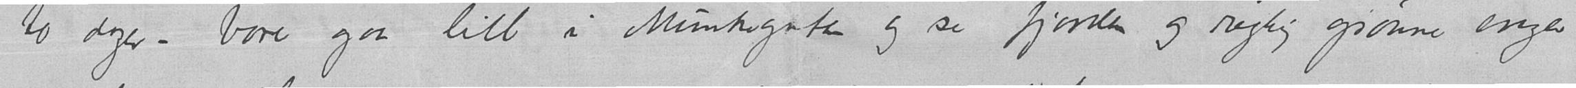to dager – bare gaa litt i Munkegaten og se fjorden og rigtig grønne enger
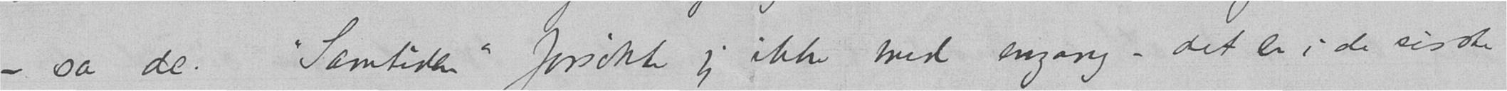– sa de. "Samtiden" forsøkte jeg ikke med engang – det er i de sisste
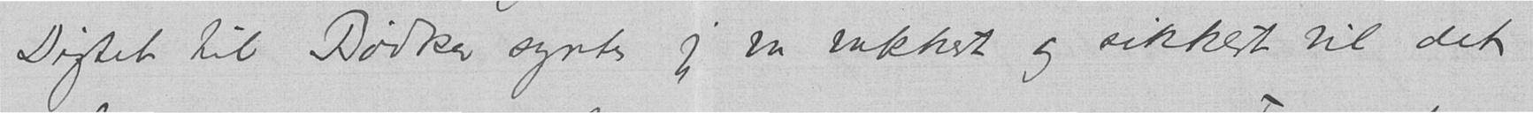Digtet til Bødker syntes jeg var vakkert og sikkert vil det
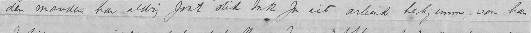den manden har aldrig faat slik tak for sit arbeid herhjemme, som han
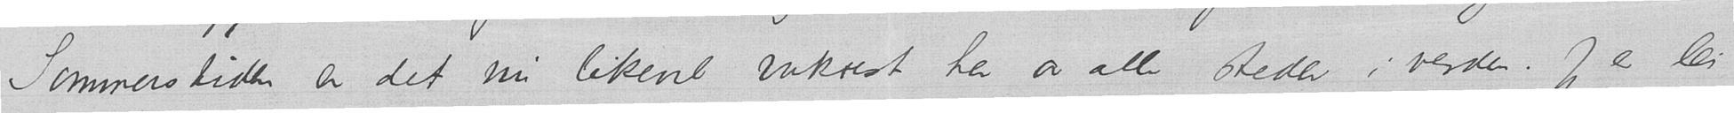Sommerstiden er det nu likevel vakrest her av alle steder i verden. Jeg er lei
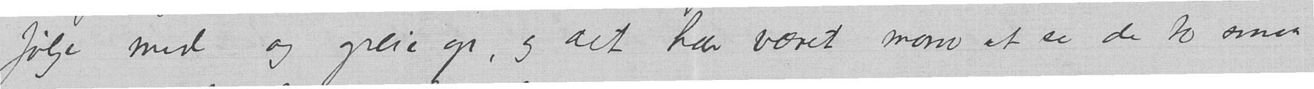følge med og greie op, og det har været morro at se de to smaa
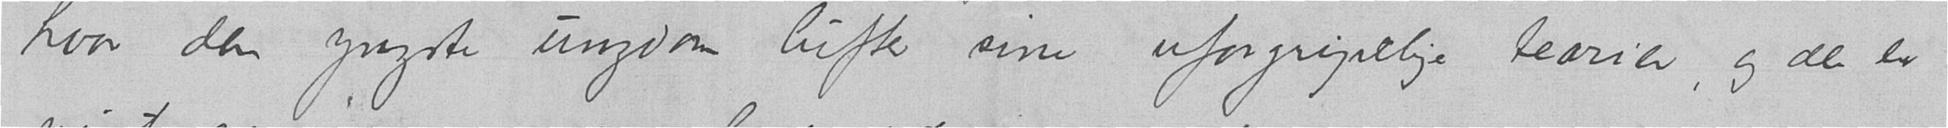hvor den yngste ungdom lufter sine uforgripelige teorier, og der er
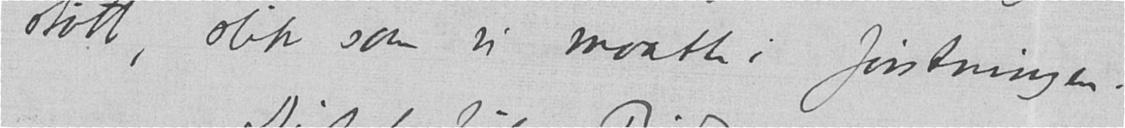støtt, slik som vi maatte i førstningen.
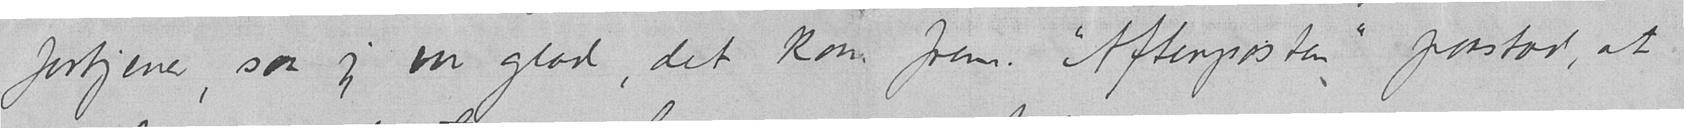fortjener, saa jeg var glad, det kom frem.  "Aftenposten" paastod, at
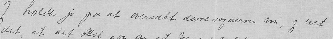Jeg holder jo paa at oversætte disse sagaerne nu, jeg vet
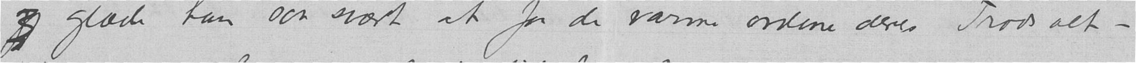gj glæde ham saa svært at faa de varme ordene deres. Trods alt –
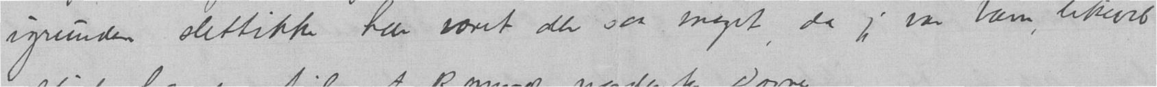igrunden slettikke har været der saa meget da jeg var barn, likevel
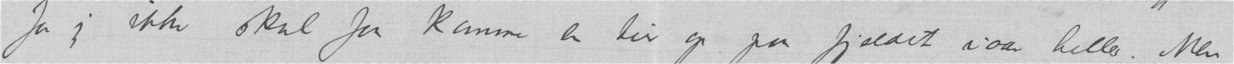for jeg ikke skal faa komme en tur op paa fjeldet iaar heller. Men
